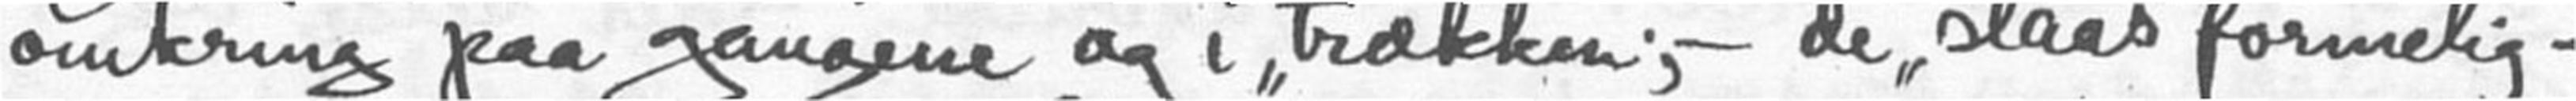omkring paa gangene og i "trækken"; -de "slaas" formelig -
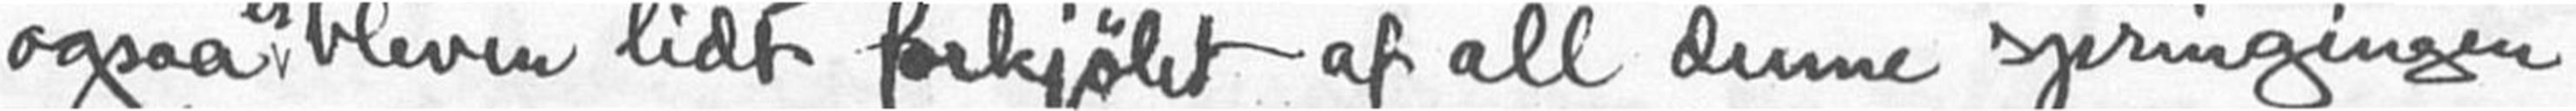ogsaa er bleven lidt forkjølet af all denne springingen
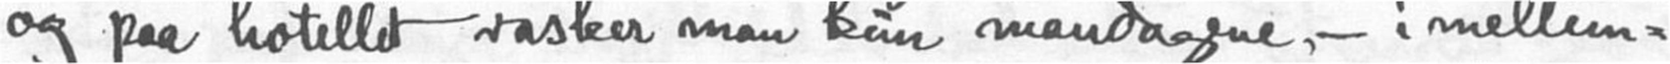og paa hotellet vasker man kun mandagene, - i mellem-
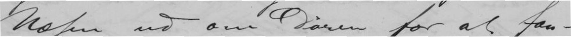Næsen ud om Døren for at fan-
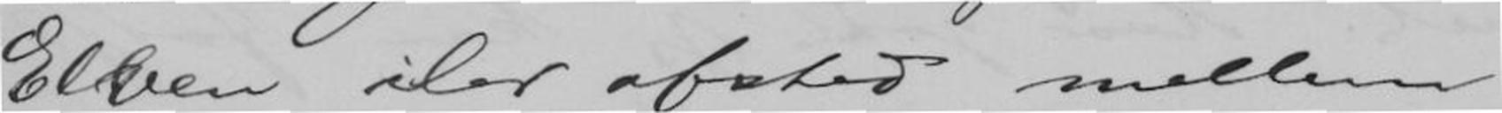Elven iler afsted mellem
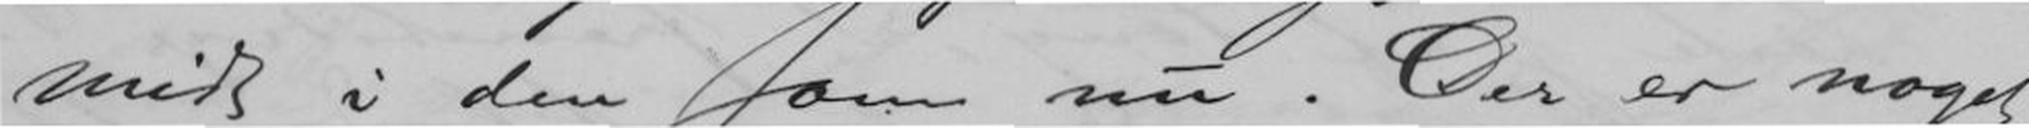midt i den som nu. Der er noget
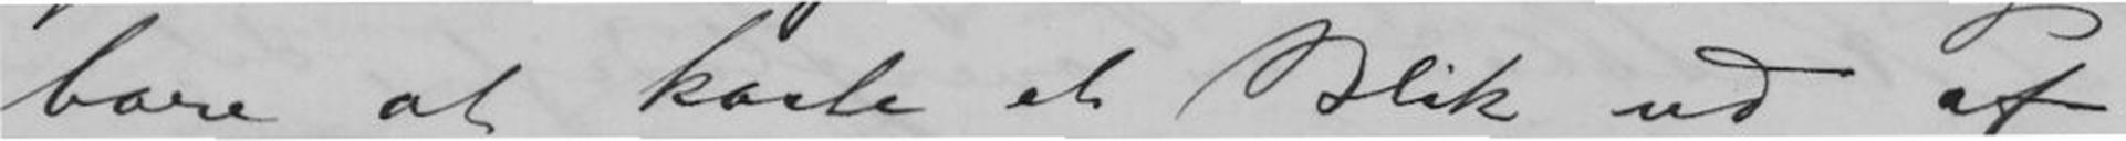bare at kaste et Blik ud af
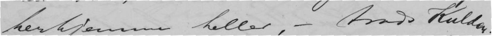herhjemme heller, - trods Kulden.
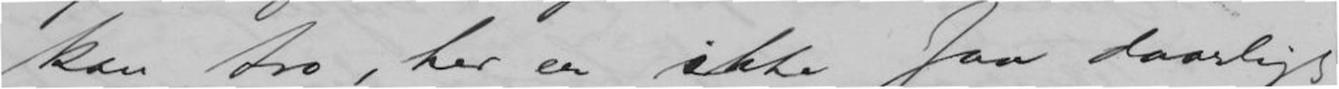kan tro, her er ikke saa daarligt
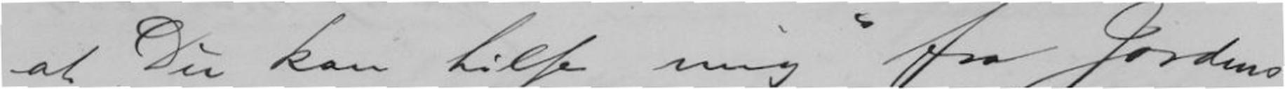at Du kan hilse mig fra Jordens
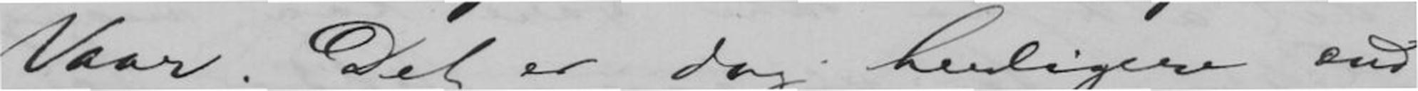Vaar. Det er dog herligere end
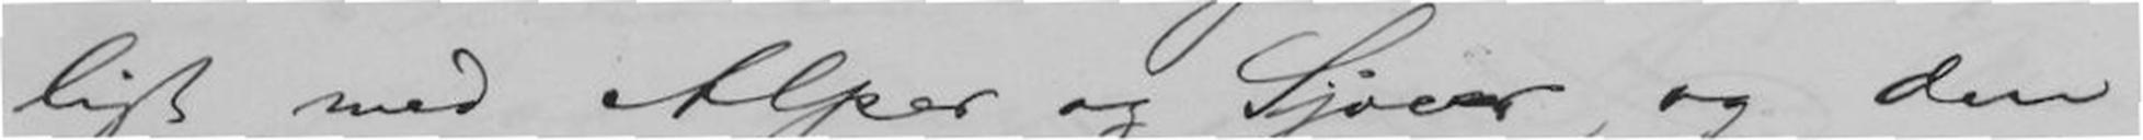ligt med Alper og Sjøer, og den
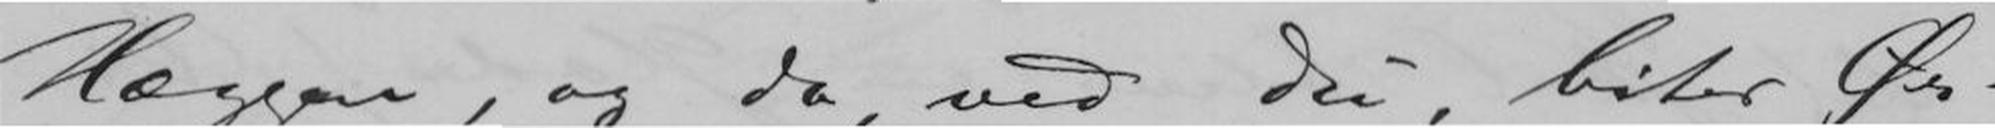Hæggen, og da, ved Du, biter Ør-
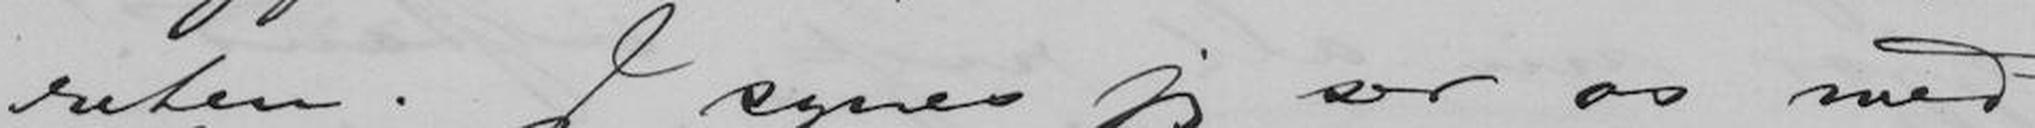reten. Jeg synes jeg ser os med
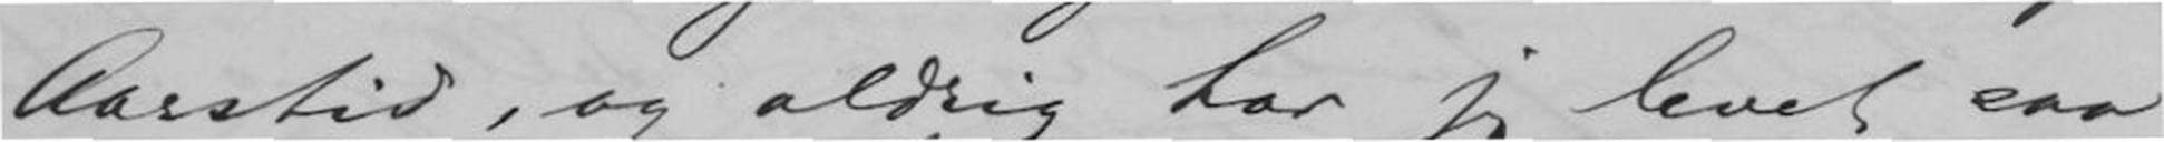Aarstid, og aldrig har jeg levet saa
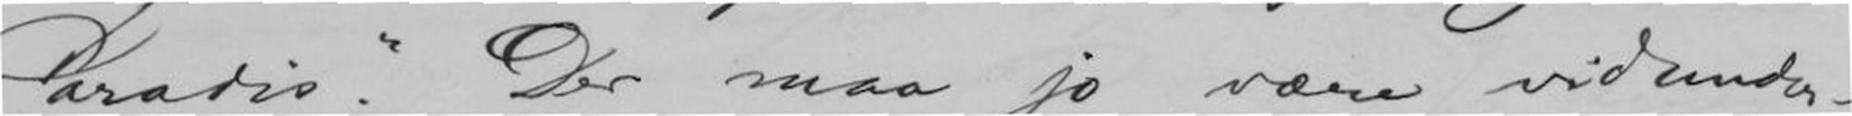Paradis. Der maa jo være vidunder-
